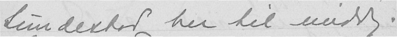Lundestad her til middag.
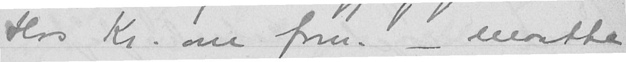Hos Kr. om form. - maatte
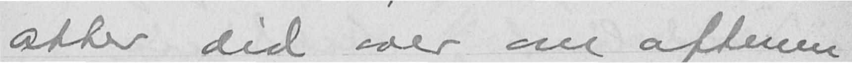atter did over om aftenen
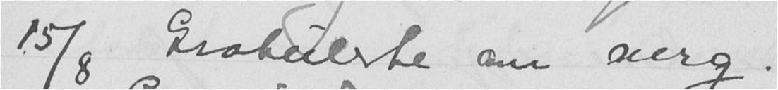15/8 Gratulerte om mrg.
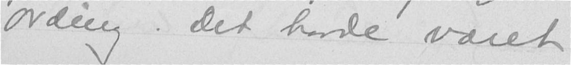Ording. Det havde været
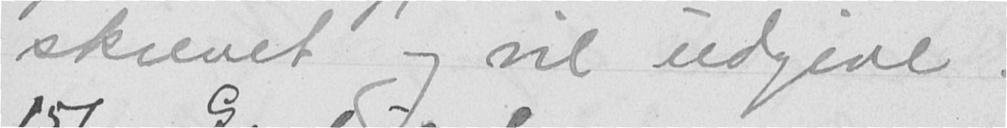skrevet og vil udgive.
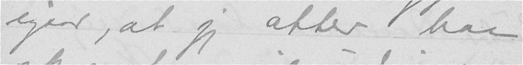igaar, at jeg atter har
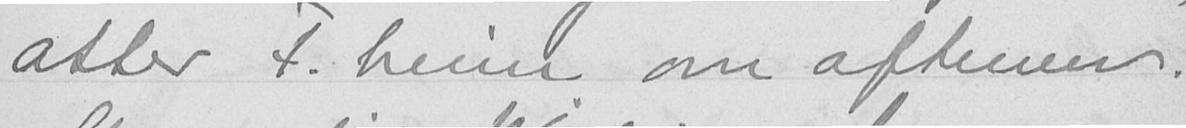atter F. heim om aftenen.
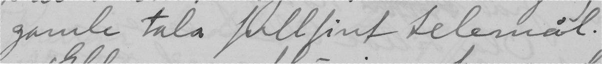gamle tala fullfint telemål.
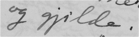og gjilde.
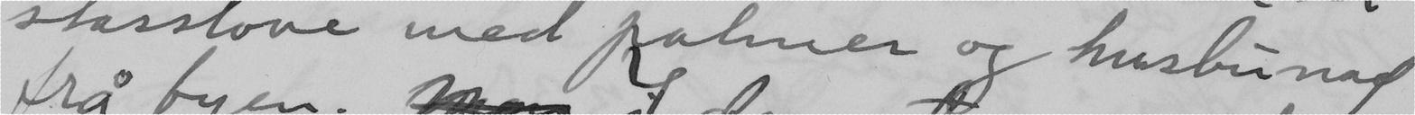stasstove med palmer og husbunad
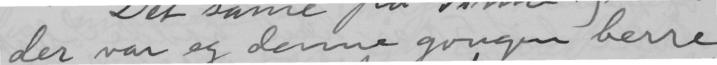der var eg denne gongen berre
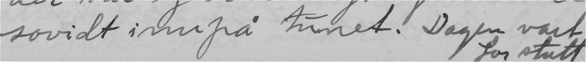sovidt innpå tunet. Dagen vart
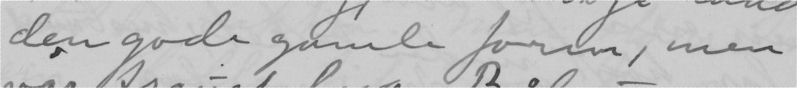den gode gamle form, men
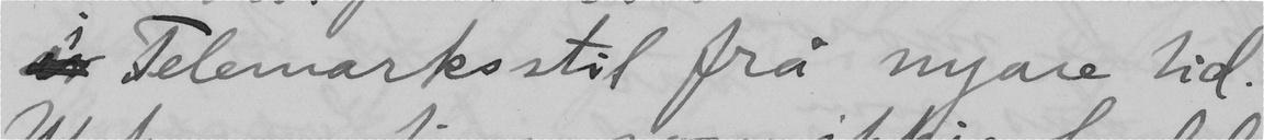i Telemarksstil frå nyare tid.
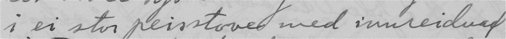i ei stor peisstove med inureidnad
